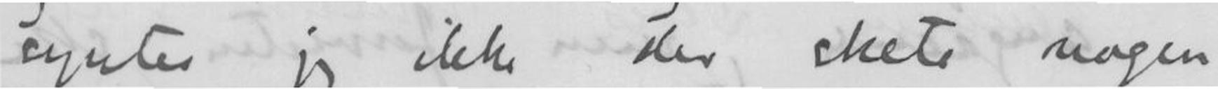syntes jeg ikke der skete nogen
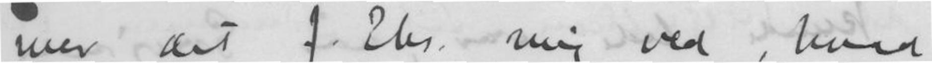mer det f. Eks. mig ved, hvad
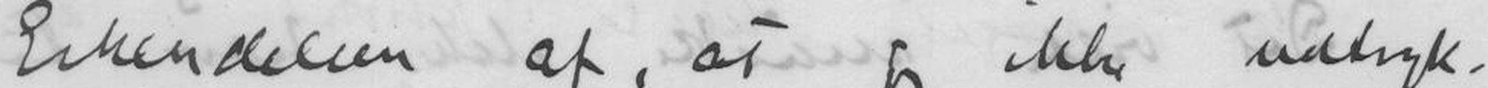Erkendelsen af, at jeg ikke udtryk-
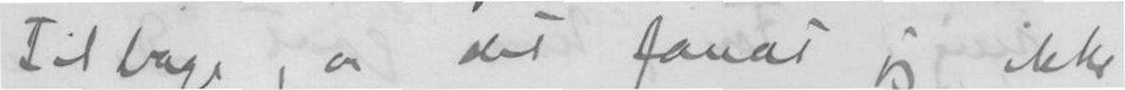tilbage, og det fandt jeg ikke
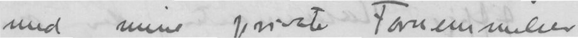med mine private Fornemmelser
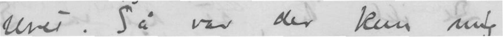Uret. Så var der kun mig
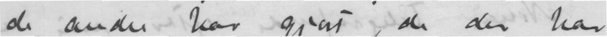de andre har gjort, de der har
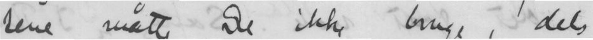tene Måtte De ikke bruge, dels
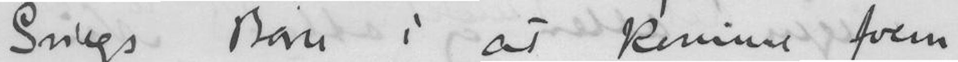Griegs Børn i at komme frem
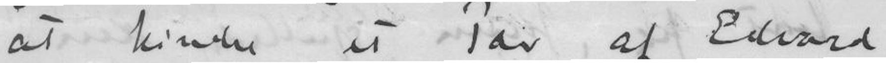at hindre et Par af Edvard
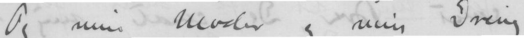Og min Moder og min Dreng
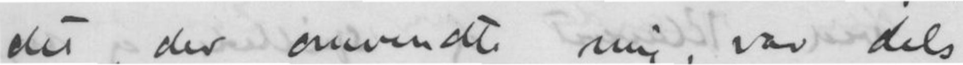du, der omvendte mig, var dels
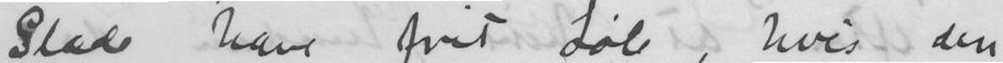Glæde have frit Løb, hvis den
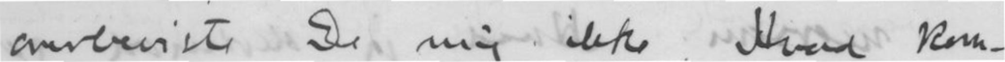overbeviste De mig ikke. Hvad kom-
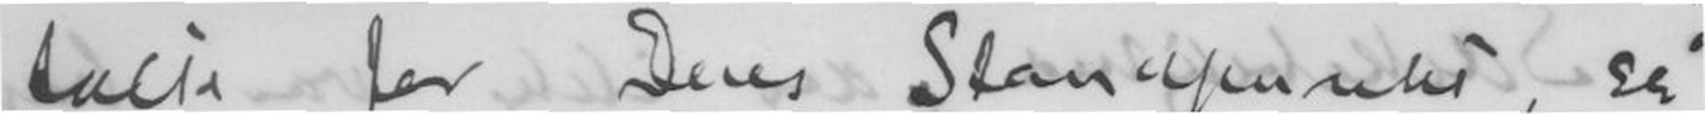talte for Deres Standpunkt, så
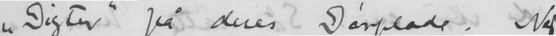Digter på deres Dørplade. Nej,
